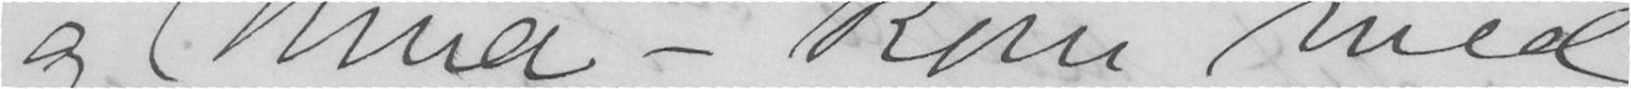og Nina - kom med
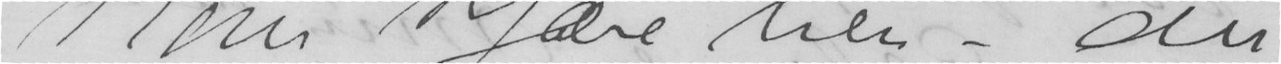Kom kjære ven - du
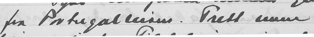fra Portugal igjen. Trett men
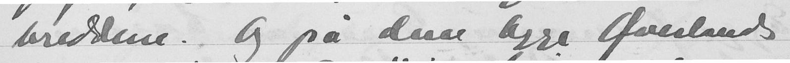breddene. Og på dem begge Øverlands
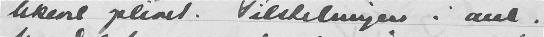likevel oplivet. Tilstelningen i anl.
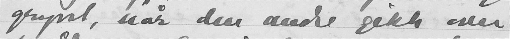grynt, når den andre gikk over
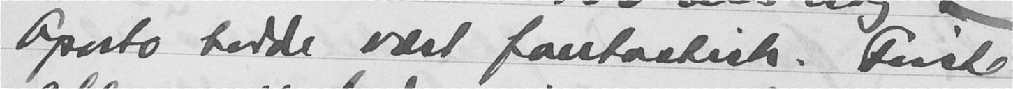Opsto hadde vært fantastisk. Siste
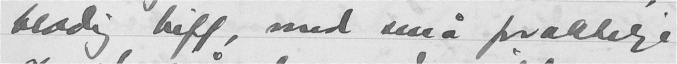blodig biff, med små foraktelige
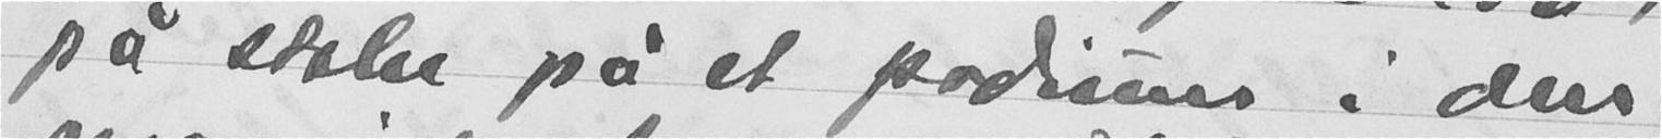på stolen på et podium i den
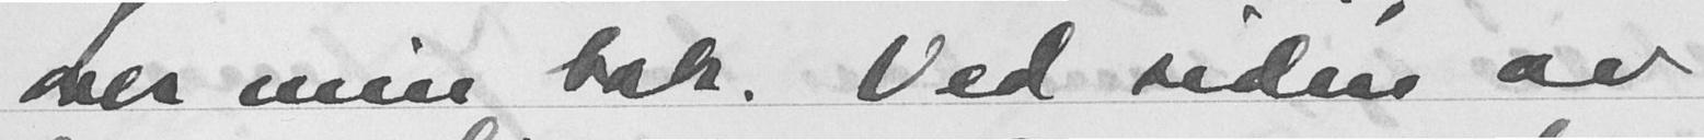over min bok. Ved siden av
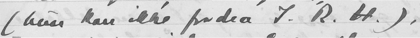(hun kan ikke fordra J. R. H.),
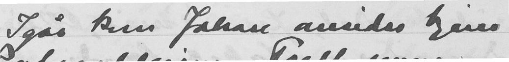Igår kom Johan omsider hjem
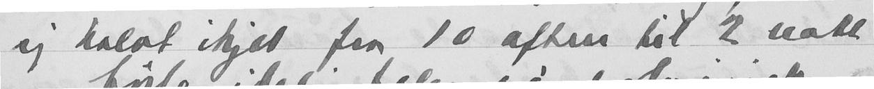sig halvt ihjel fra 10 aften til 2 natt
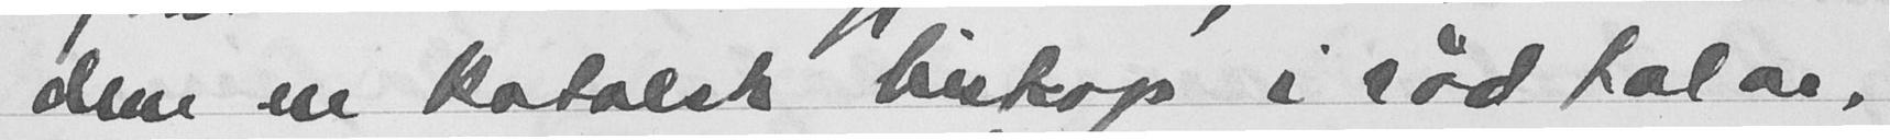dem en katolsk biskop i rød kalas,
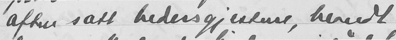aften satt hedersgjesterne, blandt
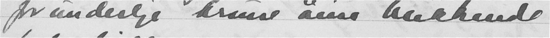forunderlige brune øine kikkende
